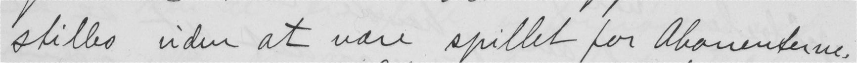stilles uden at være spillet for Abonenterne.
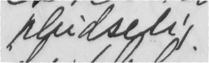pludselig
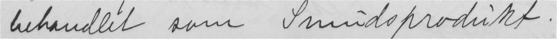behandlet som Smudsprodukt.
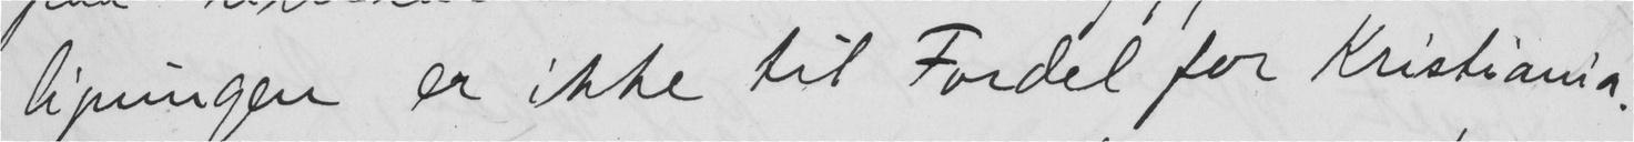ligningen er ikke til Fordel for Kristiania.
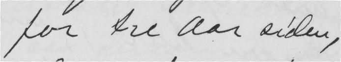for tre Aar siden,
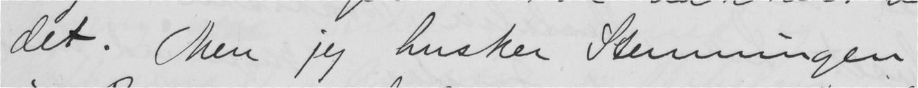det. Men jeg husker Stemningen
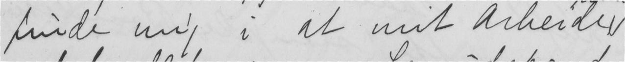fiude mig i at mit Arbeide
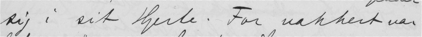sig i sit Hjerte. For vakkert var
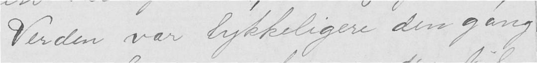Verden var lykkeligere den gang
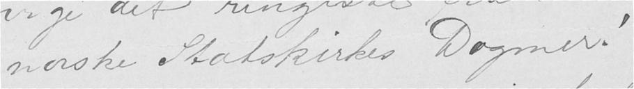norske Statskirkes Dogmer!
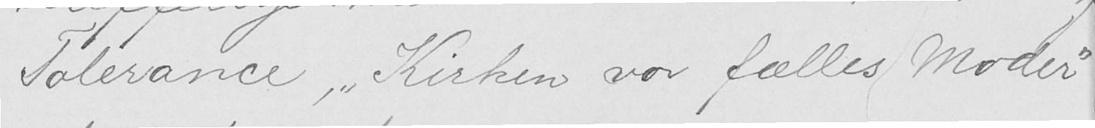Tolerance, "Kirken vor fælles Moder"
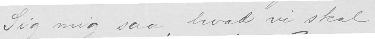Sig mig saa, hvad vi skal
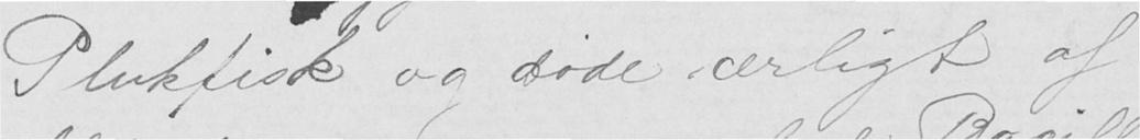Plukfisk og døde ærligt af
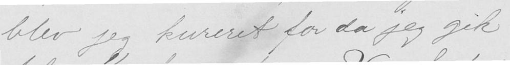blev jeg kureret for da jeg gik
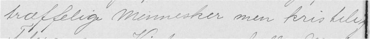træffelige Mennesker men kristelig
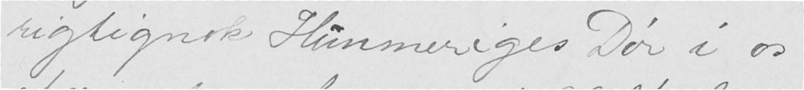rigtignok Himmeriges Dør i os
